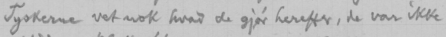Tyskerne vet nok hvad de gjør herefter, de var ikke
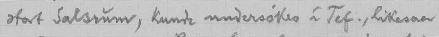stort Salsrum, kunde undersøkes i Tef., likesaa
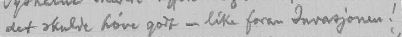det skulde høve godt - like foran Invasjonen!
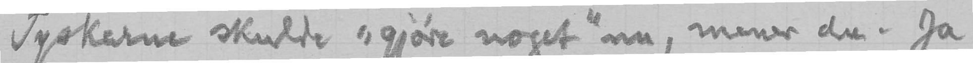Tyskerne skulde "gjøre noget" nu, mener du. Ja
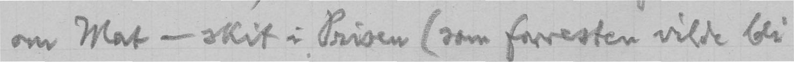om Mat - skit i Prisen (som forresten vilde bli
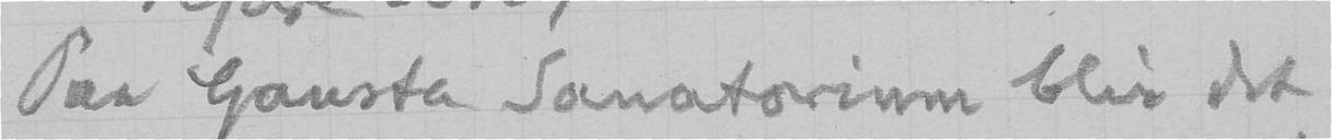Paa Gausta Sanatorium blir det
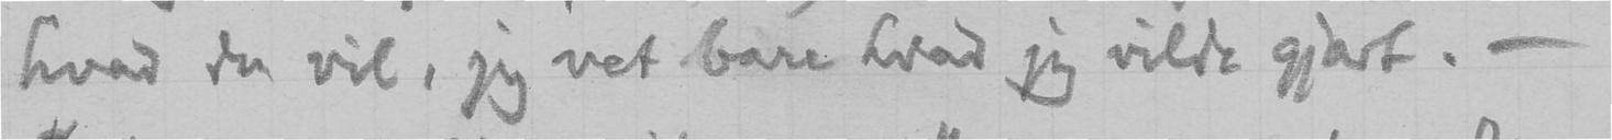hvad du vil, jeg vet bare hvad jeg vilde gjort. -
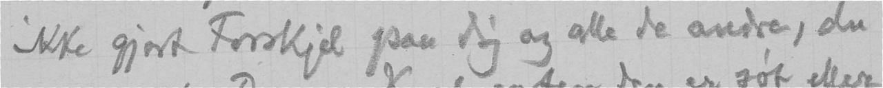ikke gjort Forskjel paa dig og alle de andre, du
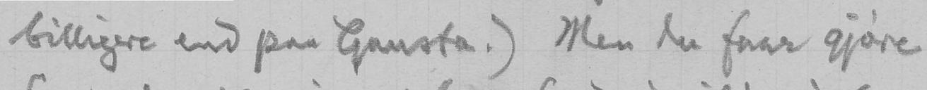billigere end paa Gausta.) Men du faar gjøre
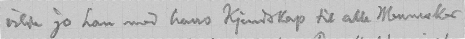vilde jo han med hans Kjendskap til alle Mennesker
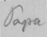Papa
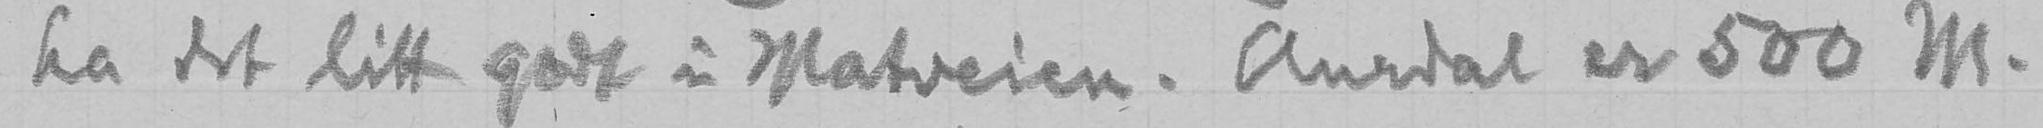ha det litt godt i Matveien. Aurdal er 500 M.
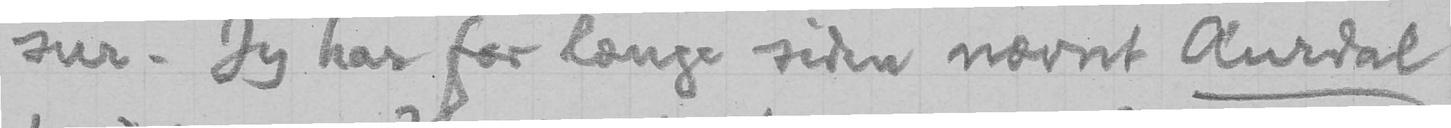sur. Jeg har for længe siden nævnt Aurdal
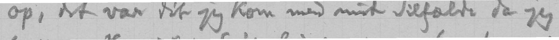op, det var dit jeg kom med mit Tilfælde da jeg
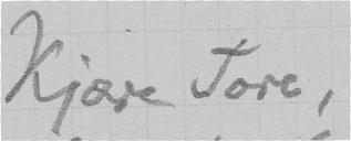Kjære Tore,
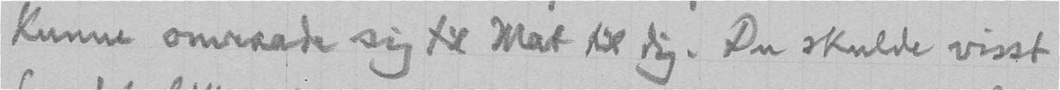kunne omraade sig til Mat til dig. Du skulde visst
